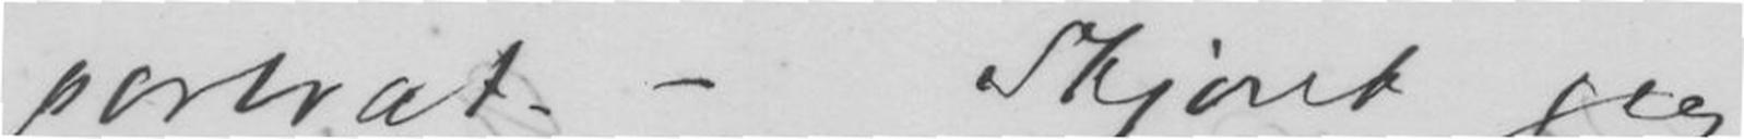portræt - Skjønt jeg
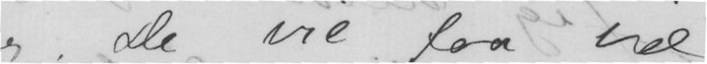jeg. De vil faa ind til
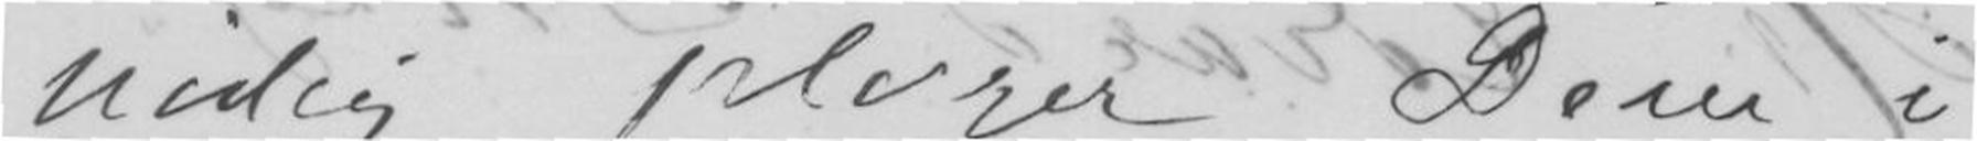nødig plager Dem i
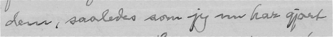dem, saaledes som jeg nu har gjort
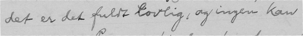det er det fuldt Lovlig, og ingen kan
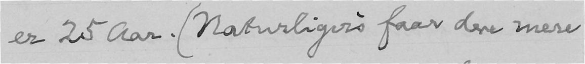er 25 Aar. (Naturligvis faar dere mere
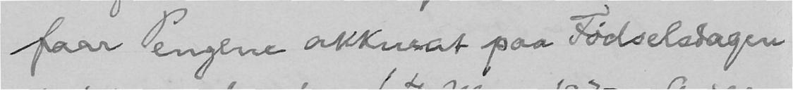faar Pengene akkurat paa Fødselsdagen
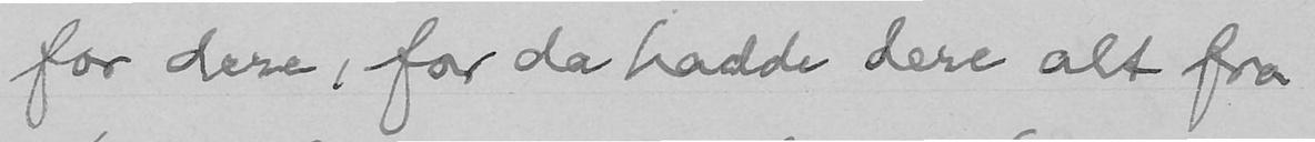for dere, for da hadde dere alt fra
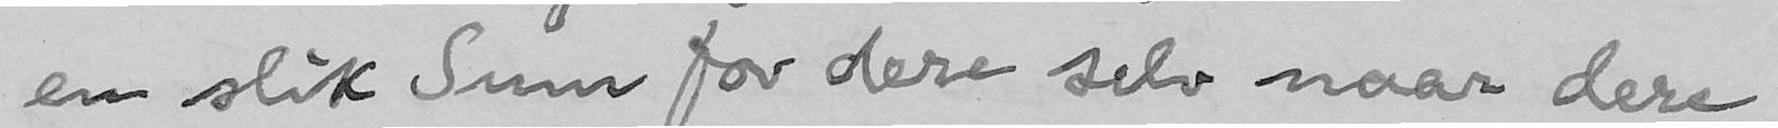en slik Sum for dere selv naar dere
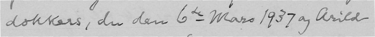dokkers, du den 6te Mars 1937 og Arild
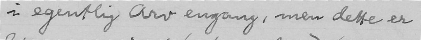i egentlig Arv engang, men dette er
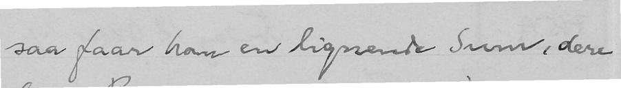saa faar han en lignende Sum, dere
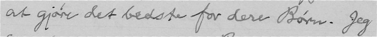at gjøre det bedste for dere Børn. Jeg
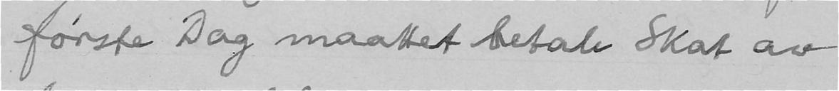første Dag maattet betale Skat av
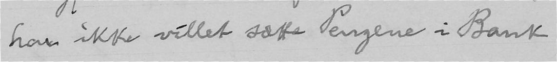har ikke villet sætte Pengene i Bank
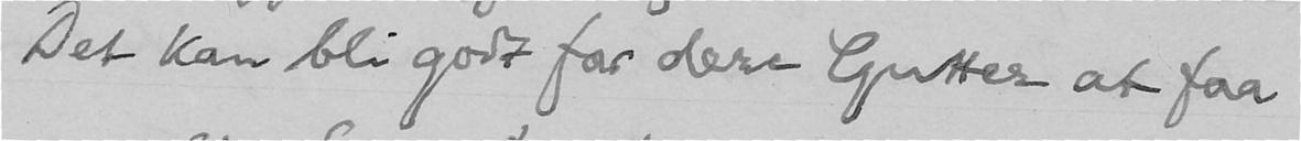Det kan bli godt for dere Gutter at faa
